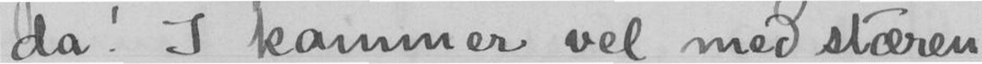da! I kommer vel med stæren
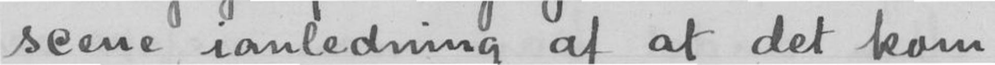scene ianledning af at det kom
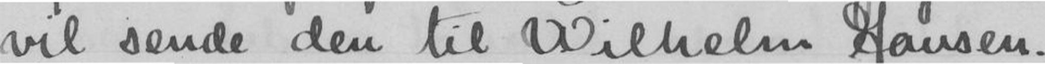vil sende den til Wilhelm Hansen.
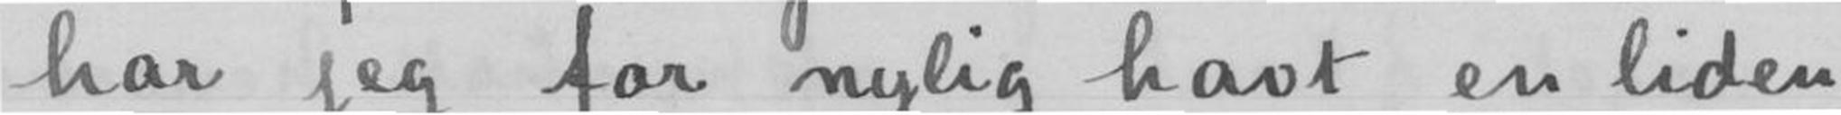har jeg for nylig havt en liden
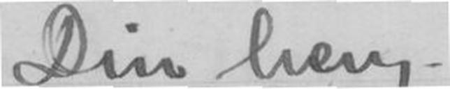Din heng.
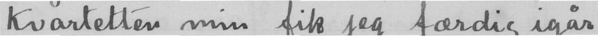Kvartetten min fik jeg færdig igår
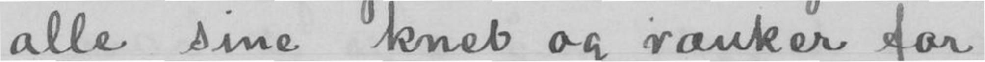alle sine kneb og rænker for
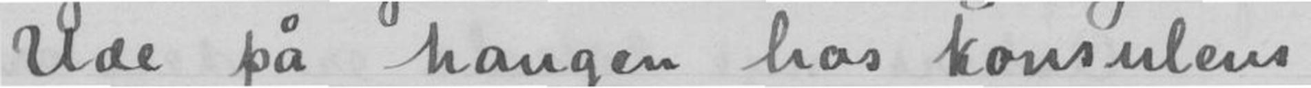Ude på haugen hos konsulens
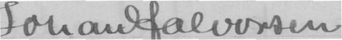Johan Halvorsen
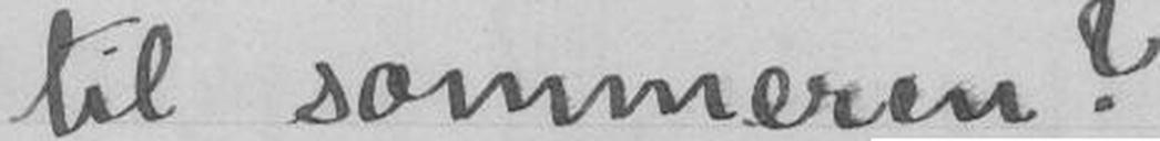til sommeren?
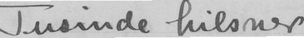Tusinde hilsner
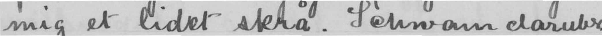mig et lidet skrå. Schwam daruber.
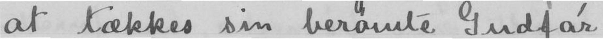at tækkes sin berømte Gudfa'r
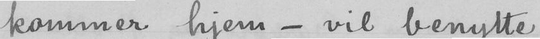kommer hjem - vil benytte
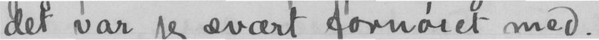det var jeg svært fornøiet med.
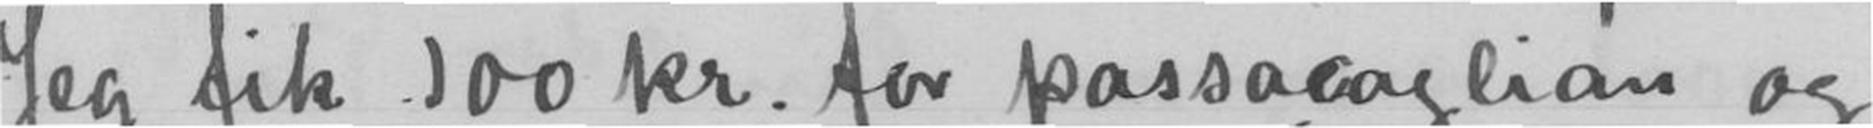Jeg fik 100 kr. for passacaglian og
